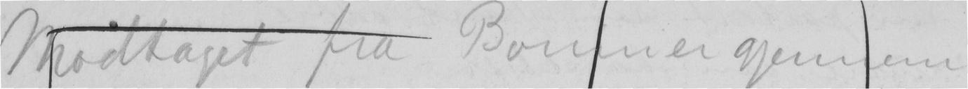Modtaget fra Bonnier gjennem
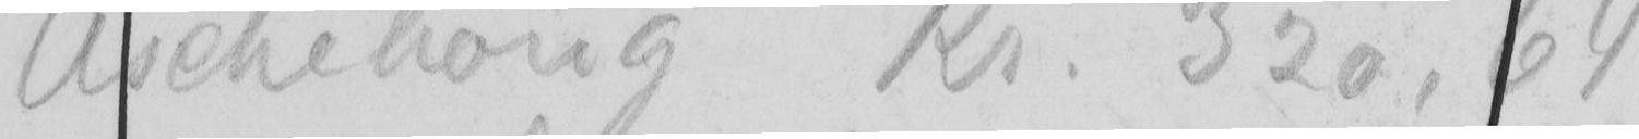Aschehoug Kr. 320,64
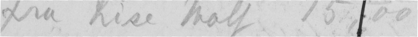fra Lise Wolf 15,00
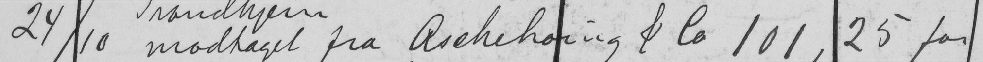24/10 modtaget fra Aschehoug & Co 101,25 for
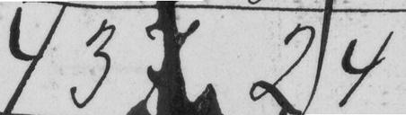437,24
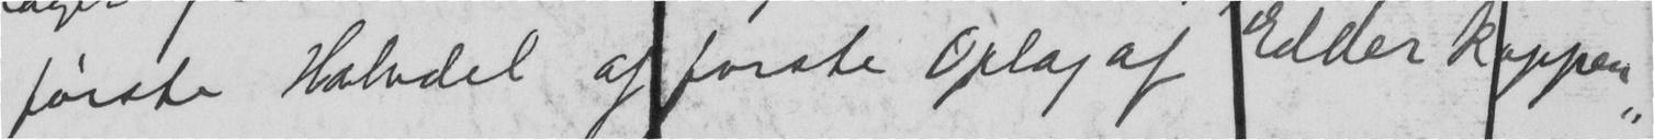første Halvdel af første Oplag af "Edderkoppen"
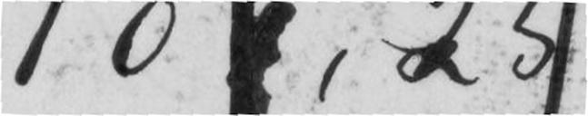101,25
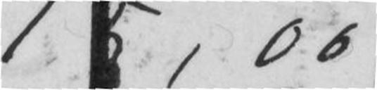15,00
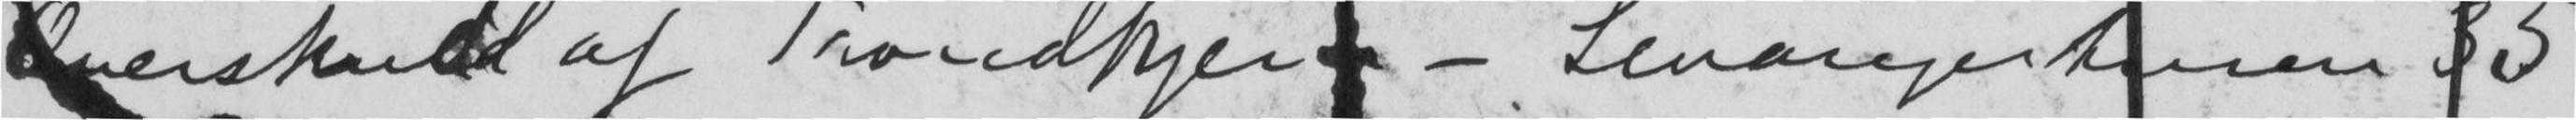Overskudd af Trondhjem - Levangerturen 35
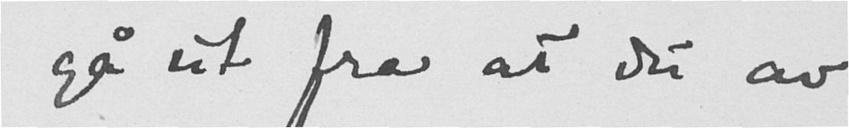gå ut fra at du av
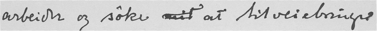arbeider og søke mit at tilveiebringe
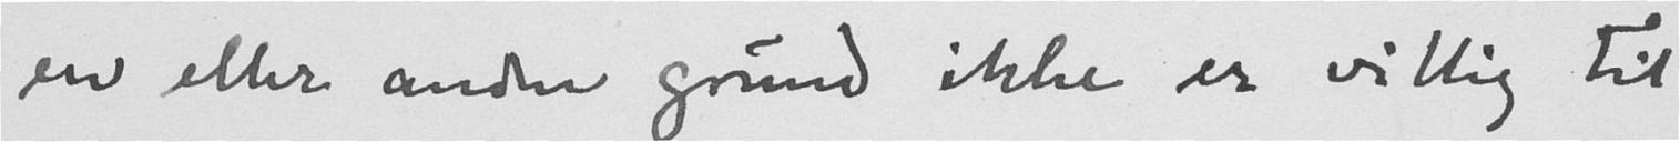en eller anden grund ikke er villig til
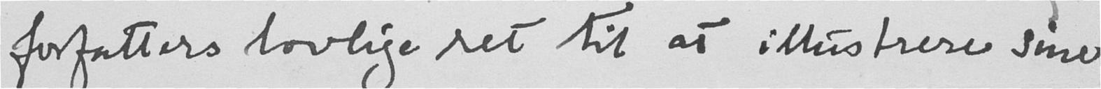forfatters lovlige ret til at illustrere sine
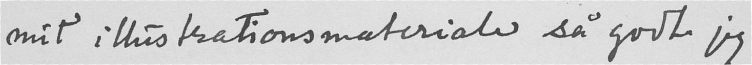mit illustrationsmateriale så godt jeg
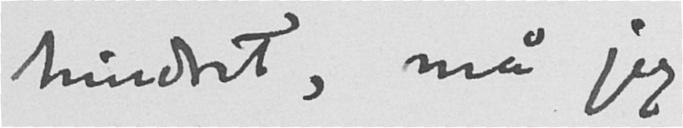hindret, må jeg
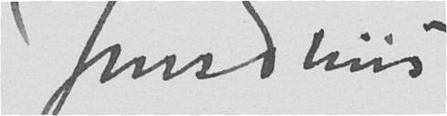Jens Thiis
